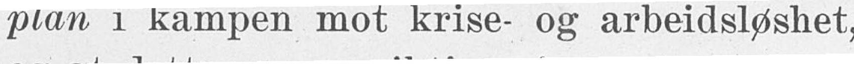plan i kampen mot krise- og arbeidsløshet,
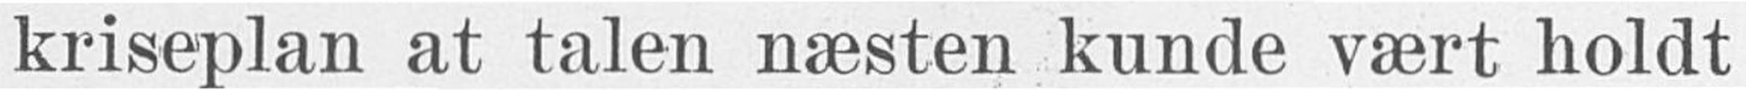kriseplan at talen næsten kunde vært holdt
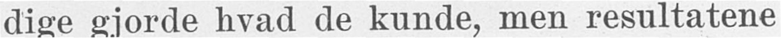dige gjorde hvad de kunde, men resultatene
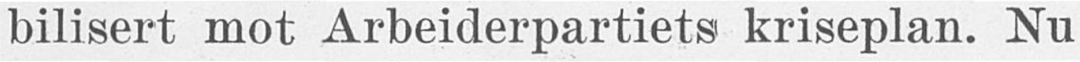bilisert mot Arbeiderpartiets kriseplan. Nu
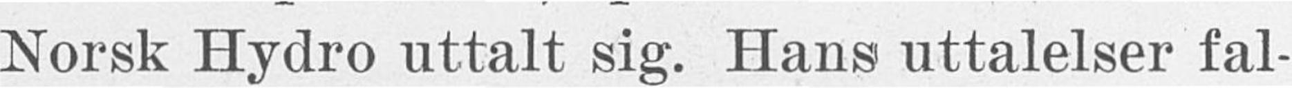Norsk Hydro uttalt sig. Hans uttalelser fal-
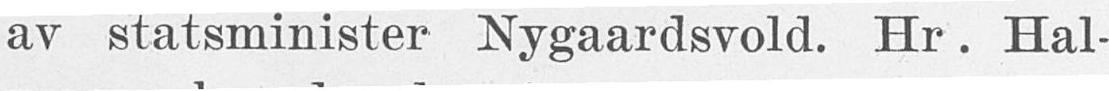av statsminister Nygaardsvold. Hr. Hal-
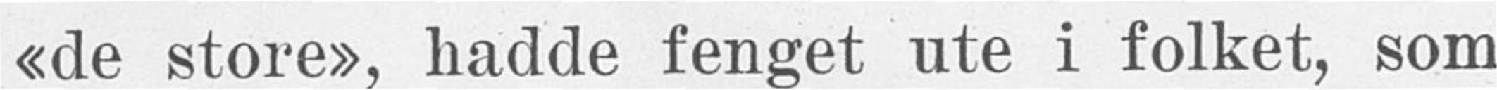«de store», hadde fenget ute i folket, som
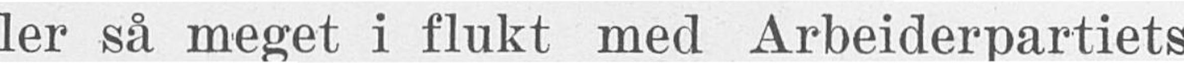ler så meget i flukt med Arbeiderpartiets
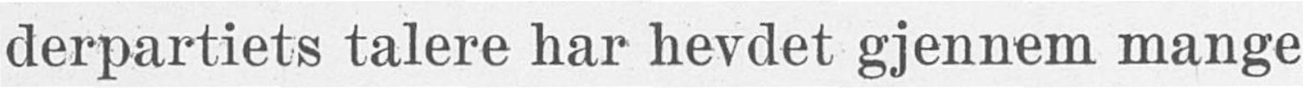derpartiets talere har hevdet gjennem mange
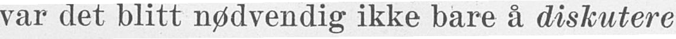var det blitt nødvendig ikke bare å diskutere
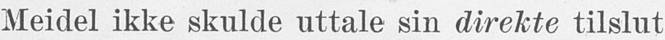Meidel ikke skulde uttale sin direkte tilslut
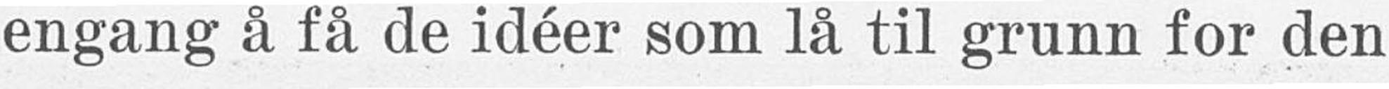engang å få de idéer som lå til grunn for den
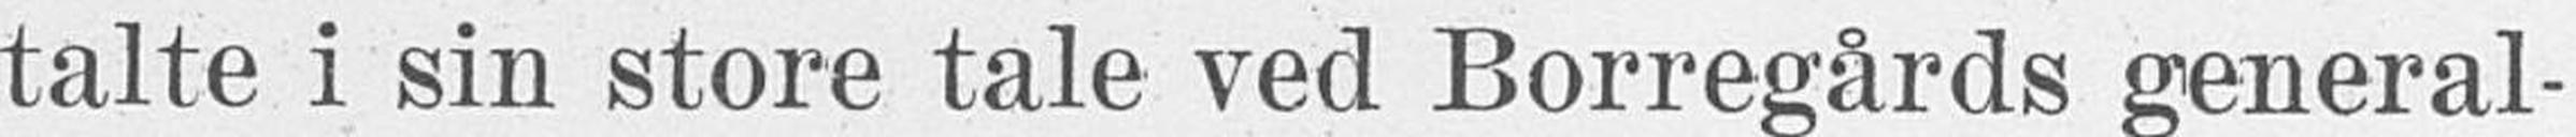talte i sin store tale ved Borregårds general-
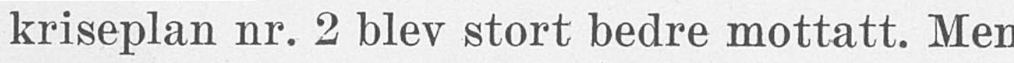kriseplan nr. 2 blev stort bedre mottatt. Men
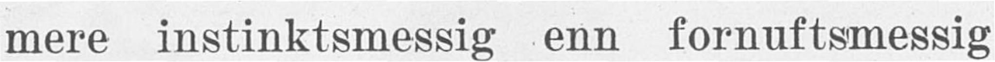mere instinktsmessig enn fornuftsmessig
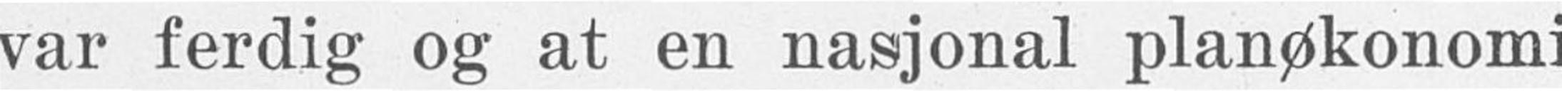var ferdig og at en nasjonal planøkonomi
